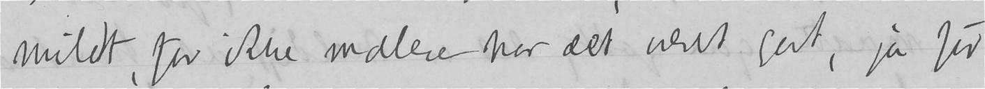mildt, for ikke malere har det været godt, ja for
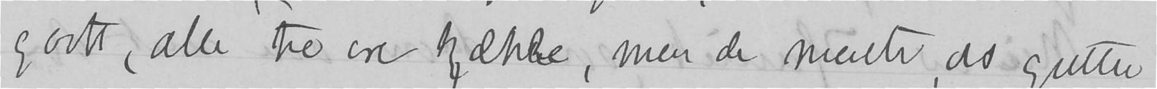godt, alle tre ere kjække, men de mente, at gutten
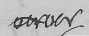verver
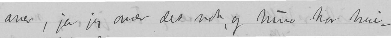aner, ja jeg aner det nok, og hun har hvi-
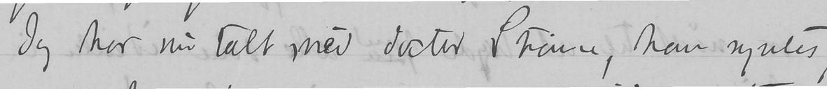Jeg har nu talt med doctor Strøm, han syntes,
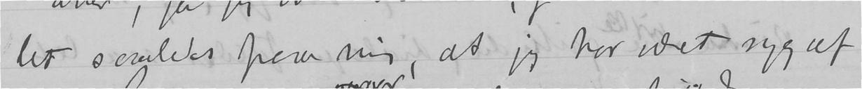let saaledes paa mig, at jeg har været syg af
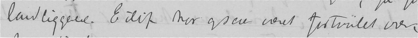landliggere. Eilif har ogsaa været fortvilet over,
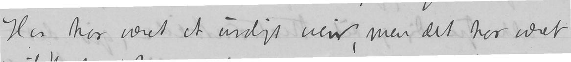Her har været et uroligt veir, men det har været
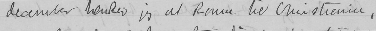december tænker jeg at komme til Christiania,
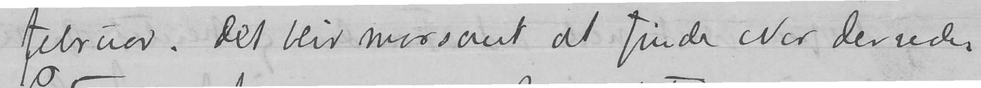februar. Det blir morsomt at finde eder derude.
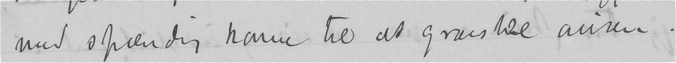med spænding komme til at granske avisen.
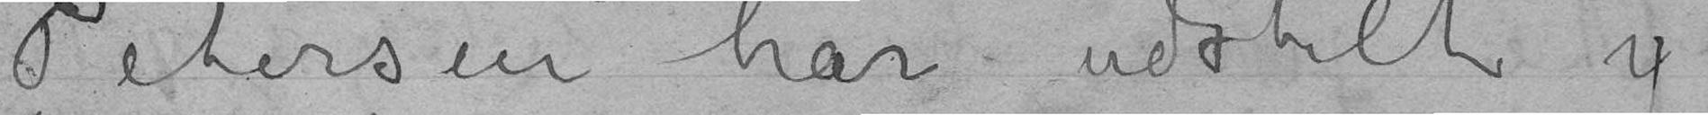Petersen har udstilt i
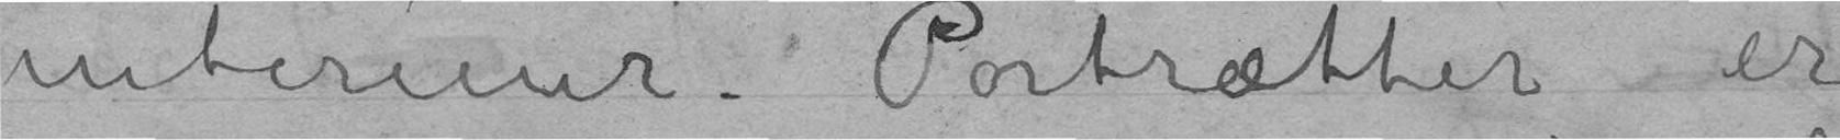interieur. Portrættet er
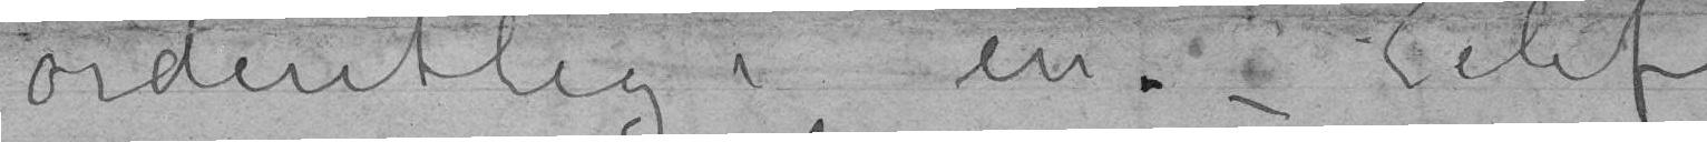ordentlig i en. Eilif
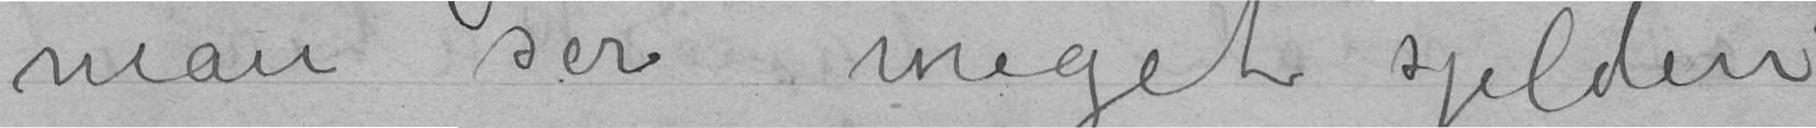man ser meget sjelden
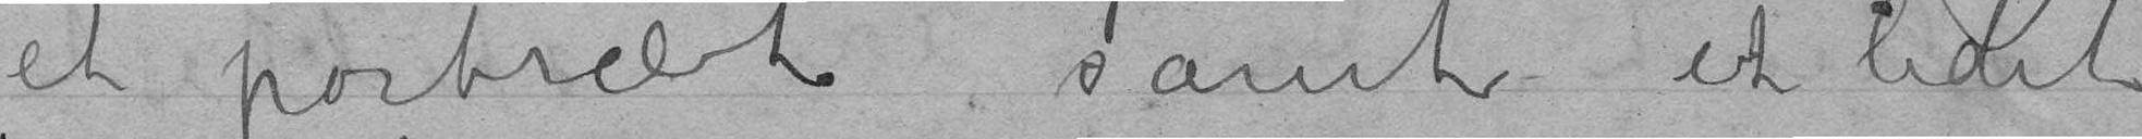et portræt samt et lidet
